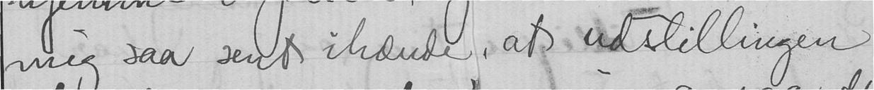mig saa sent ihænde, at udstillingen
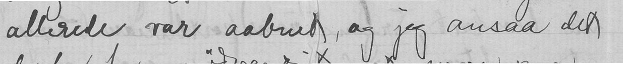allerede var aabned, og jeg ansaa det
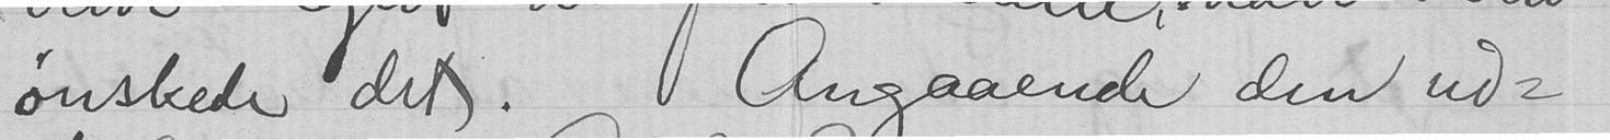ønskede det. Angaaende den ud-
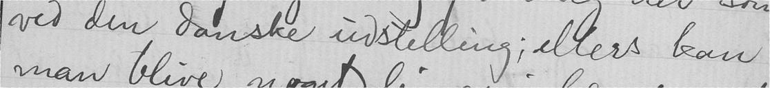ved den danske udstilling; ellers kan
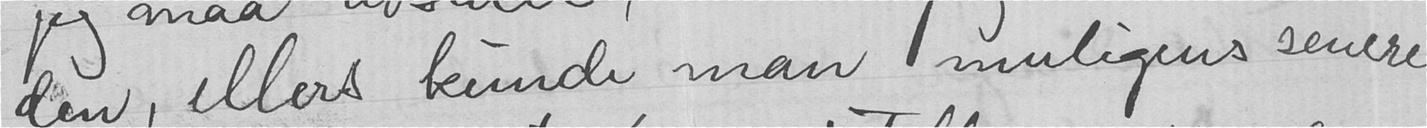den, ellers kunde man muligens senere
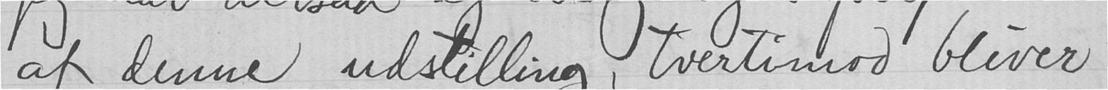af denne udstilling, tvertimod bliver
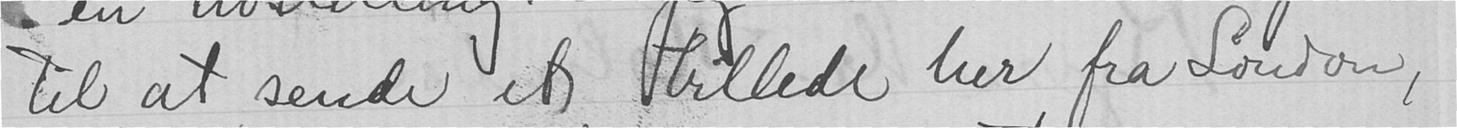til at sende et billede her fra London,
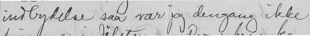indbydelse saa var jeg dengang ikke
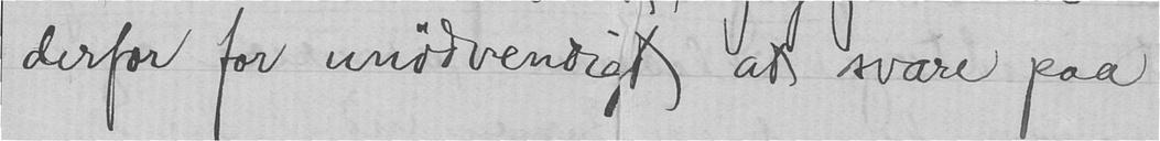derfor for unødvendigt at svare paa
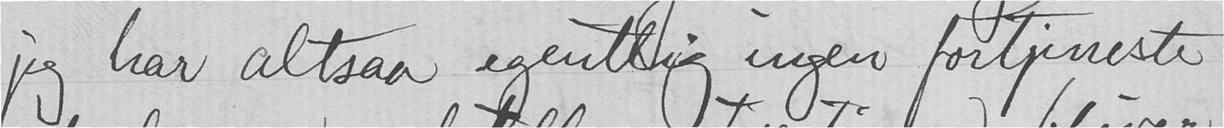jeg har altsaa egentlig ingen fortjeneste
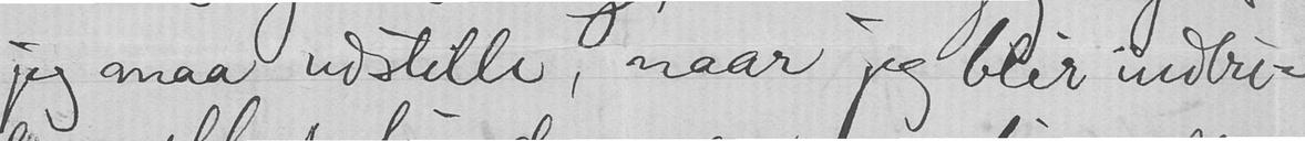jeg maa udstille, naar jeg blir indbi-
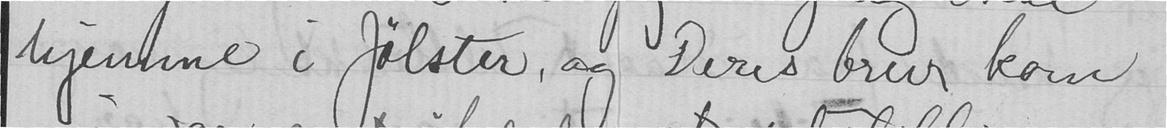hjemme i Jølster, og Deres brev kom
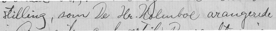stilling, som De Hr. Holmboe arangerede
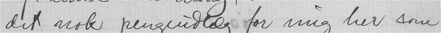det nok pengeudlæg for mig her som
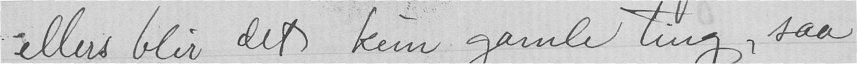ellers blir det kun gamle ting, saa
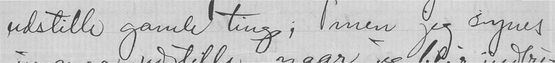udstille gamle ting; men jeg synes
